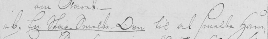"- b " En Slag-Smelte-Ovn til at smelte Ham-
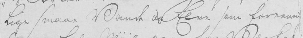lige smaae Vande og Elve som foreene
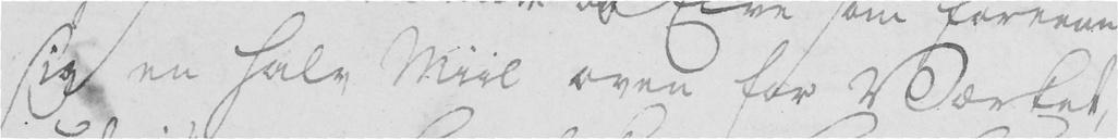sig en halv Miil oven for Værket,
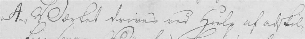"- 4 -" Værket drives ved Hielp af adskil-
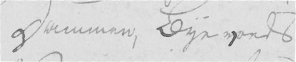Dammen, Bye vands
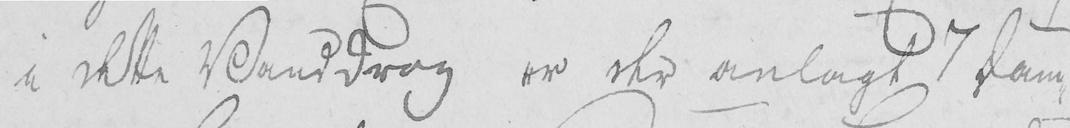i dette Vanddrag er der anlagt 7 Dam-
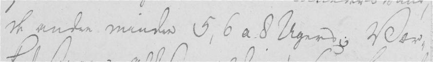de andre mindre 5, 6 a 8 Uger; Vær-
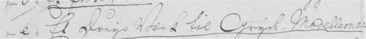"- e " Et Dreye Værk til Gryde-Modellerne
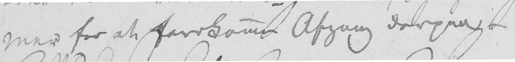mer for at forekome Afgang derpaa -
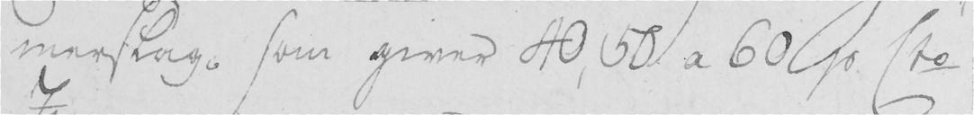merslag; som giver 40, 50 a 60 jo Eto
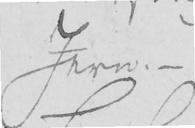Jern. -
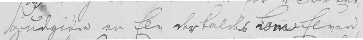og udgiør en Elv der kaldes Lom-Elven,
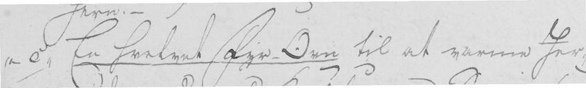"- c " En hvelvet Fyr-Ovn til at varme Jer-
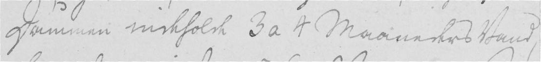Dammen indeholde 3 a 4 Maaneders Vand,
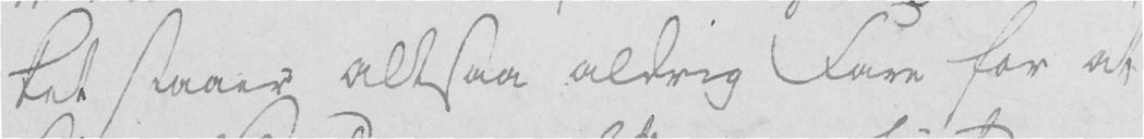ket staaer altsaa aldrig Fare for at
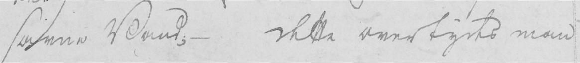savne Vand; - Dette overtydes man
